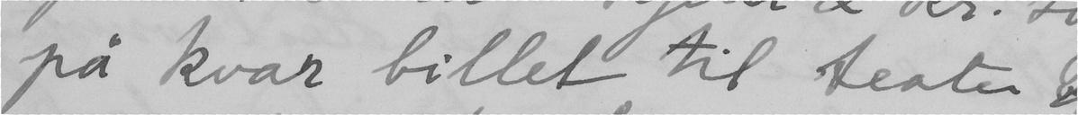på kvar billet til teater
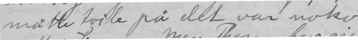måtte tvile på det var noko
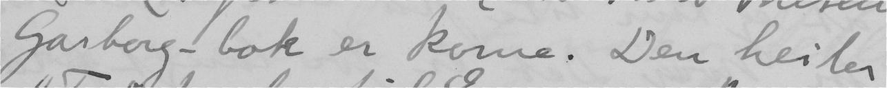Garborg-bok er kome. Den heiter
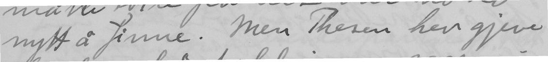nytt å finne. Men Thesen hev gjeve
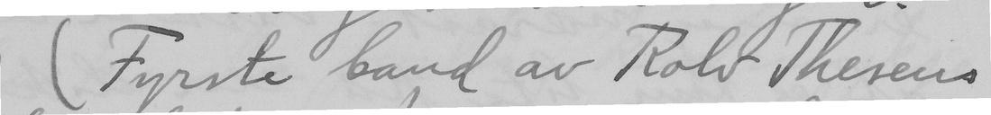Fyrste band av Rolv Thesens
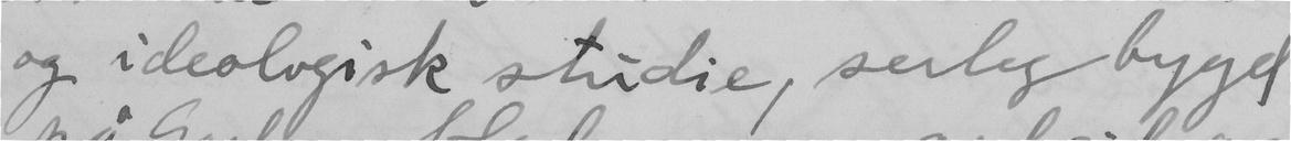og ideologisk studie, serleg bygd
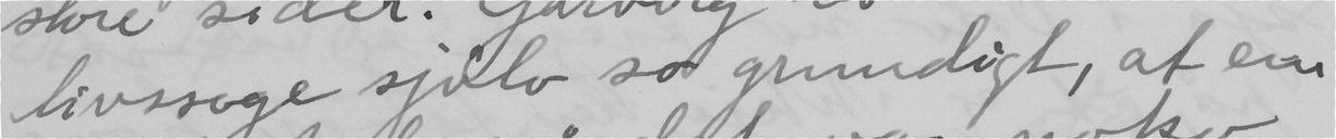livssoge sjölv so grundigt, at ein
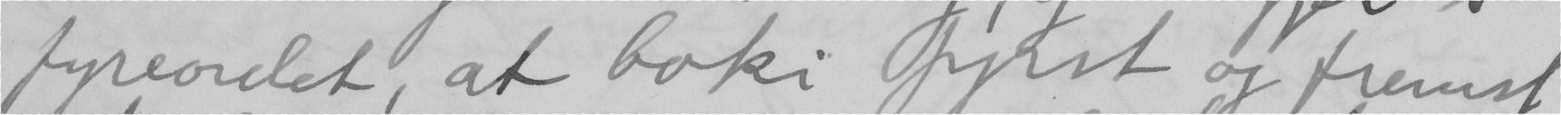fyreordet, at boki fyrst og fremst
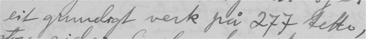eit grundigt verk på 277 tetto,
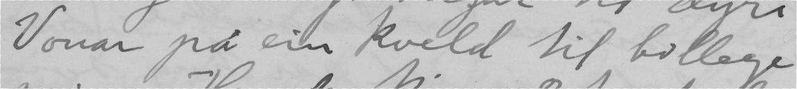Vonar på ein kveld til billege
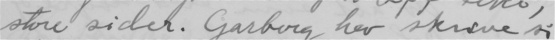store sider. Garborg hev skrive si
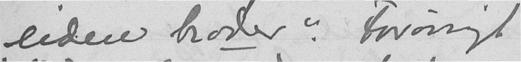liden broder. Forøvrigt
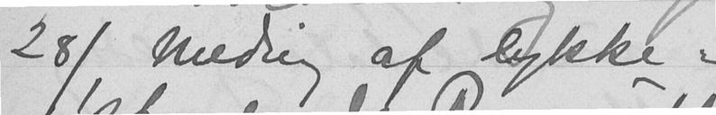28/1 Melding af lykke-
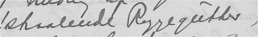straalende Roggegutter,
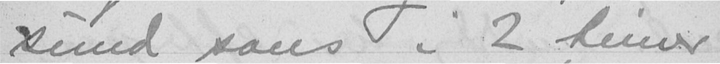sund sans i 2 timer
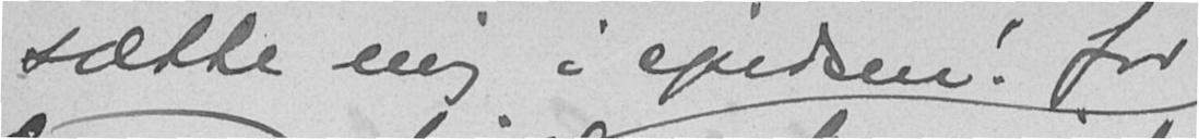sætte mig i spidsen! for
